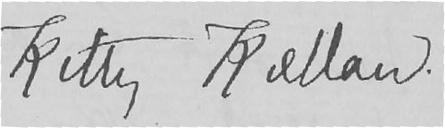Kitty Kielland.
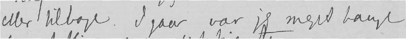eller tilbage. Igaar var jeg meget bange
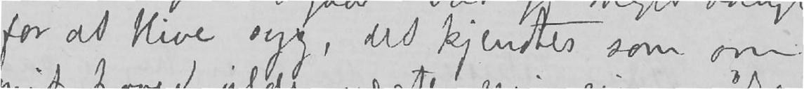for at blive syg, det kjendtes som om
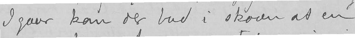Igaar kom der bud i skoven at en
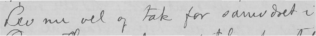Lev nu vel og tak for samværet i
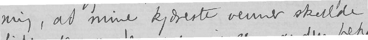mig, at mine kjæreste venner skulde
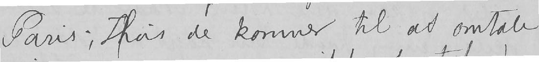Paris; Hvis de kommer til at omtale
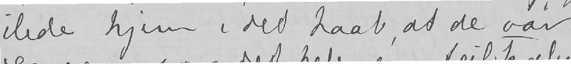ilede hjem i det haab, at de var
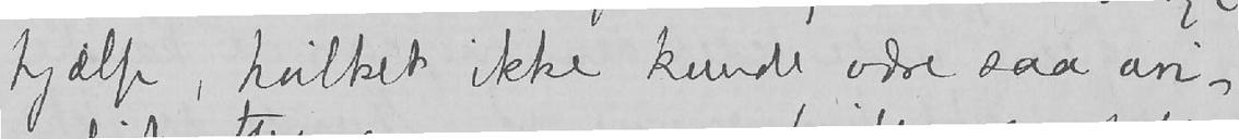hjælp, hvilket ikke kunde være saa uri-
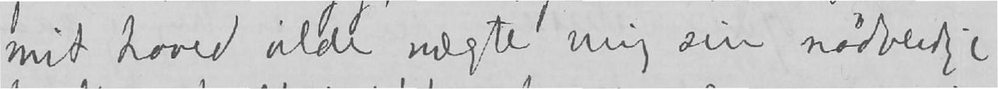mit hoved vilde nægte mig sin nødvendige
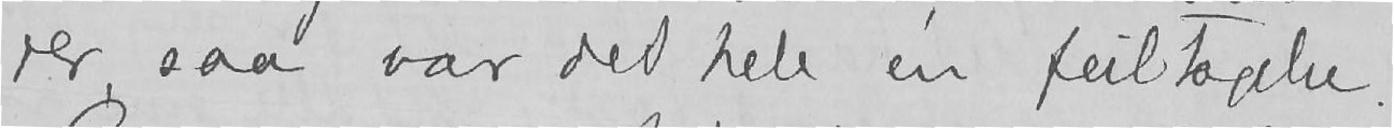der, saa var det hele en feiltagelse.
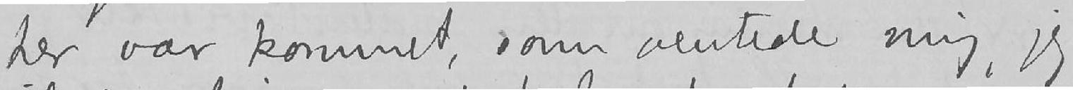her var kommet, som ventede mig, jeg
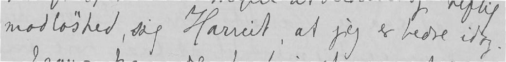modløshed, sig Harriet, at jeg er bedre idag.
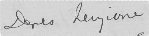Deres hengivne
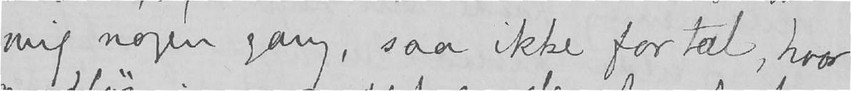mig nogen gang, saa ikke fortæl, hvor
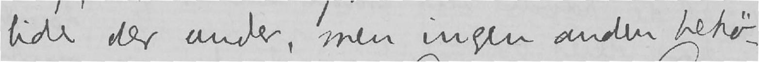lide der under, men ingen anden behø-
